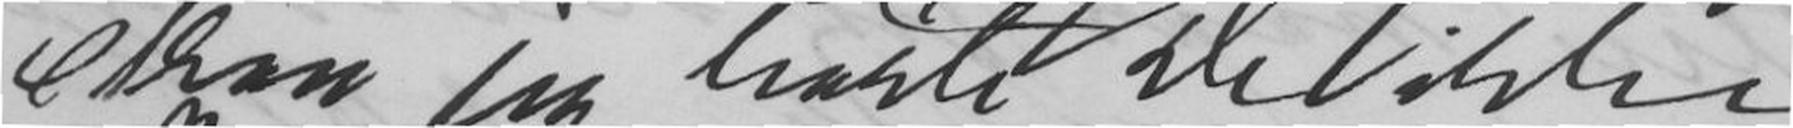strax jeg hørte De ikke
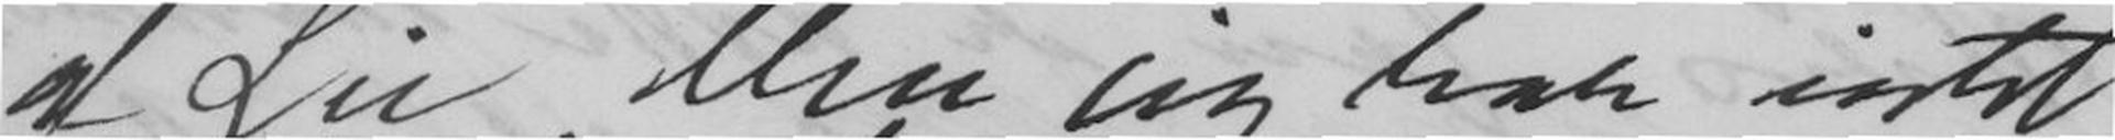af Lie. Men jeg har intet
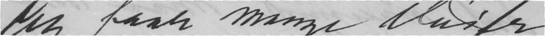jeg faar mange Møier
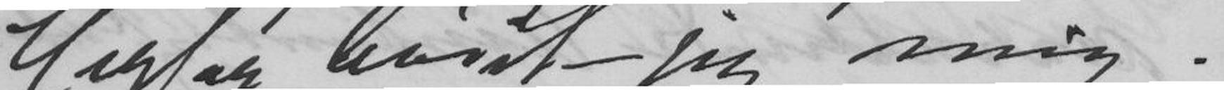Derfor høiet jeg mig.
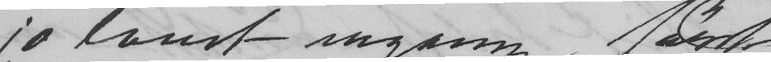jo lovet engang først
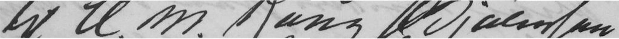til H.M. Kong Bjørnson
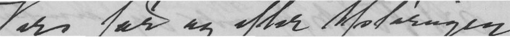Vers før og efter Afsløringen.
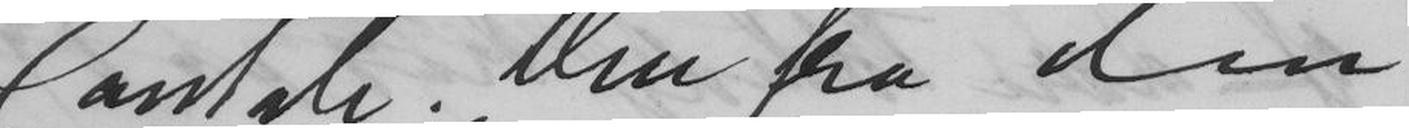Cantate. Men fra den
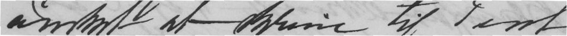ønsket at skrive til Text
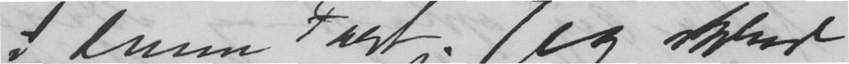i denne Fest. Jeg skrev
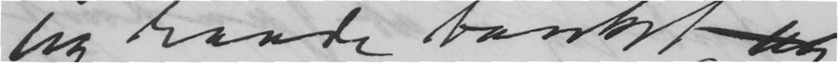jeg havde tenkt og
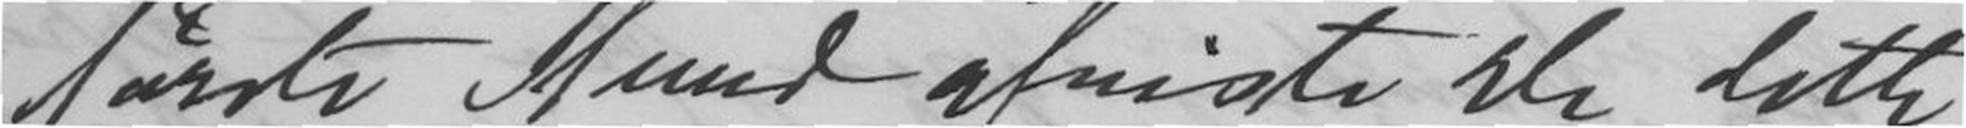første Stund afviste De dette
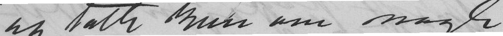og talte kun om nogle
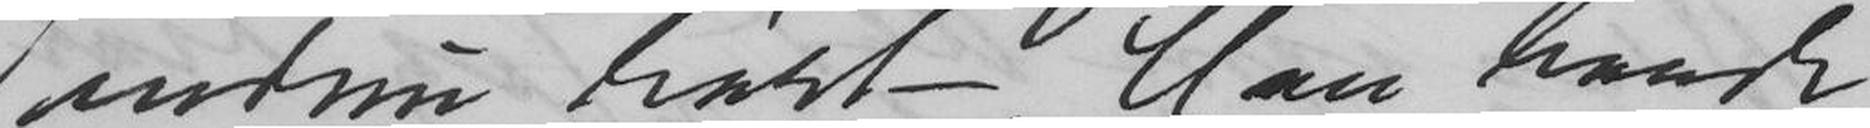endnu hørt. Han havde
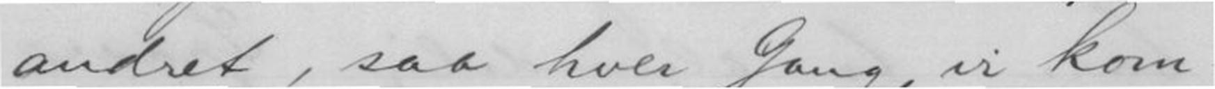andret, saa hver Gang, vi kom-
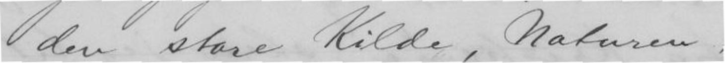den store Kilde, Naturen,
